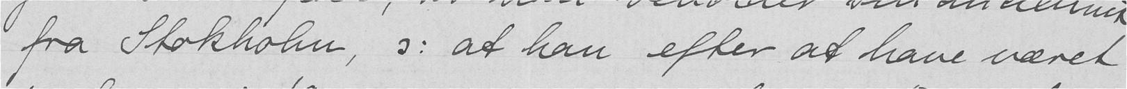fra Stokholm, : at han efter at have været
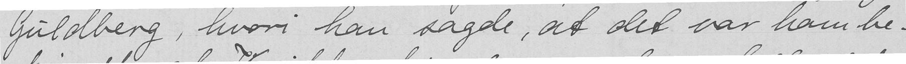Guldberg, hvori han sagde, at det var ham be-
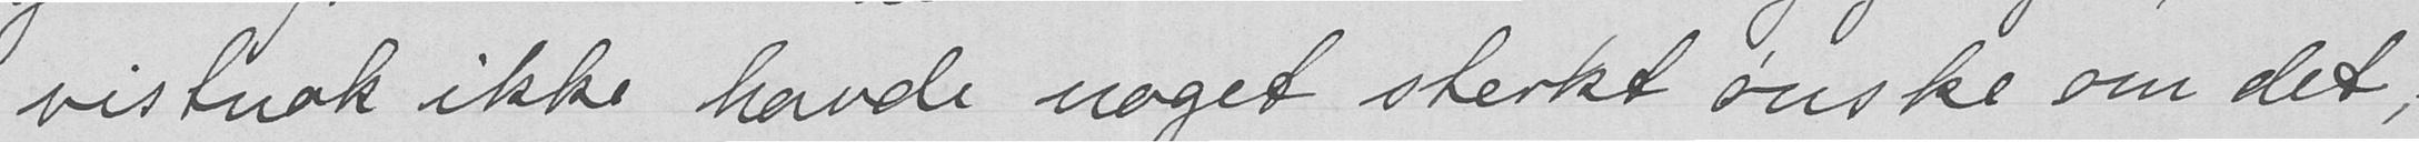vistnok ikke havde noget sterkt ønske om det.
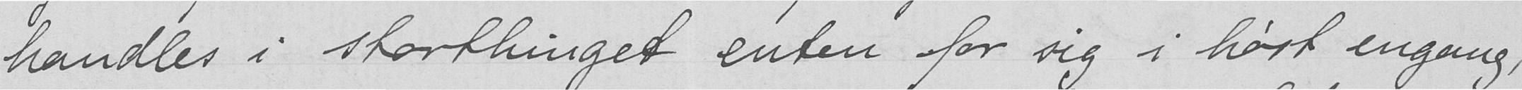handles i storthinget enten for sig i høst engang
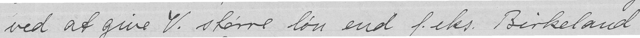ved at give V. større løn end f.eks. Birkeland
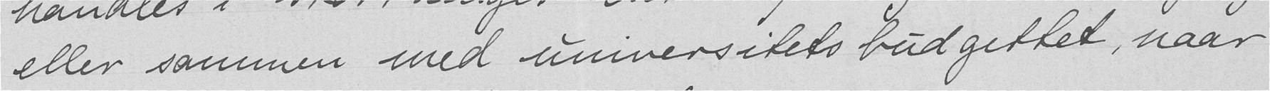eller sammen med universitetsbudgettet, naar
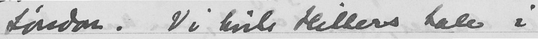Lørsda. Vi hørte Hitlers tale i
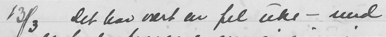13/3 - Det har vært en til uke - med
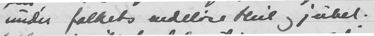under folkets endeløse Heil og jubel.
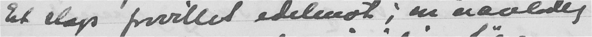Et slags forvillet edelmot; en uavladelig
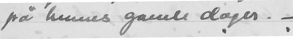på hennes gamle dager. -
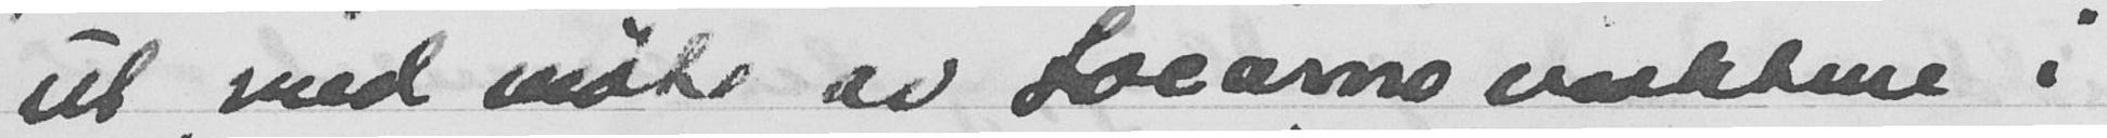ut, med møte av Loearno maktene i
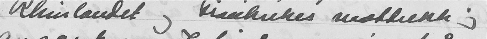Rhinlandet og Frankrikes mottrekk og
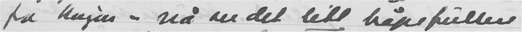fra krigen og nå ser det litt håpefullere
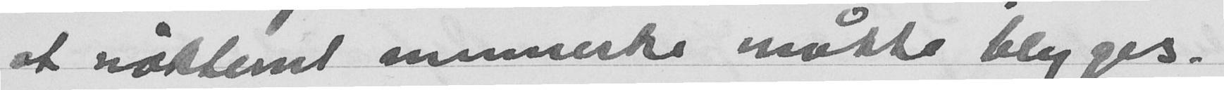at nøkternt menneske måtte blyges.
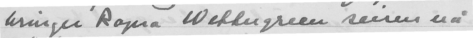bringer Ragna Wettergreen seiren nå
